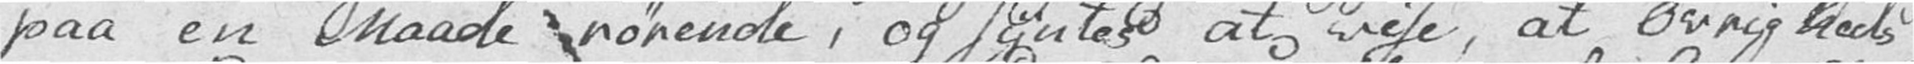paa en Maade rørende, og syntes at vise, at Øvrigheds
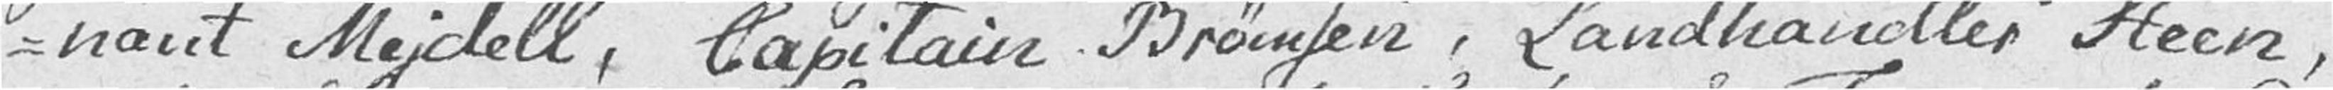-nant Mejdell, Capitain Brømsen, Landhandler Steen,
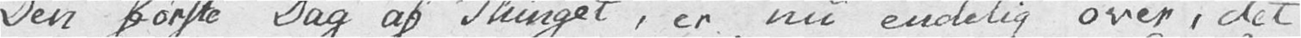Den første Dag af Thinget, er nu endelig over, det
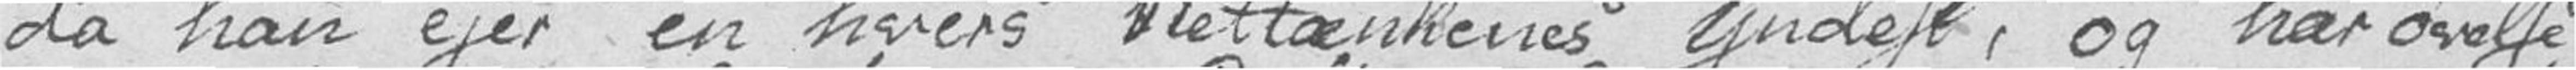da han ejer en hvers Rettænkenes Yndest, og har øvelse
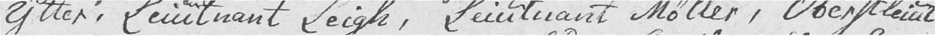Ytter, Leiutnant Leigh, Leiutnant Møller, Oberstleiut
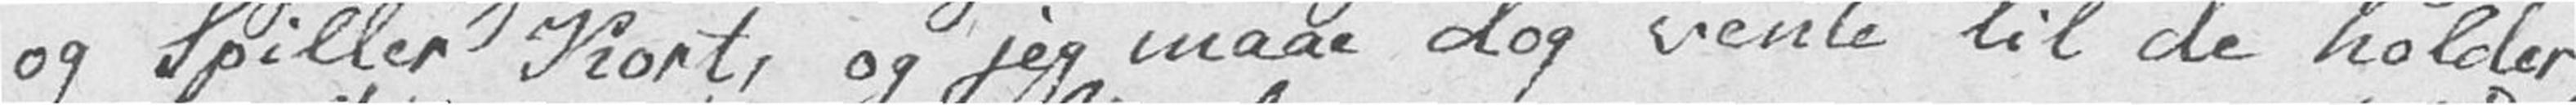og Spiller Kort, og jeg maae dog vente til de holder
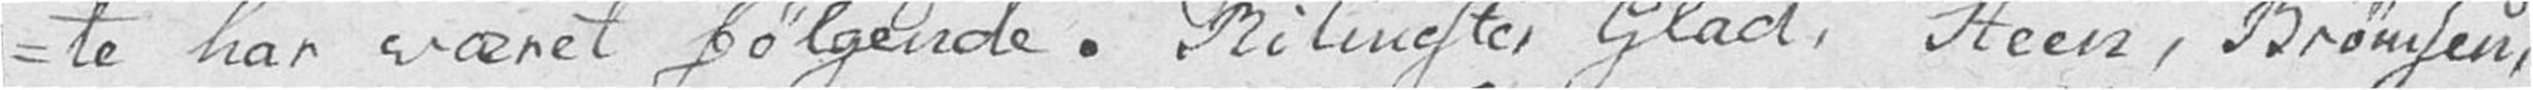-te har været følgende. Ritmester Glad, Steen, Brømsen,
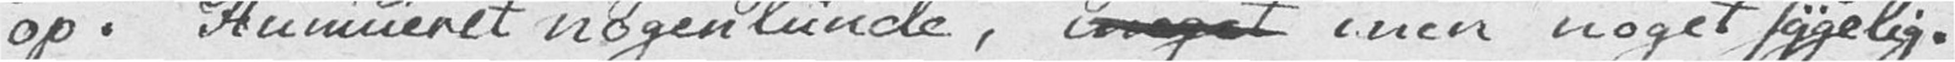op. Humueret nogenlunde, meget men noget sygelig.
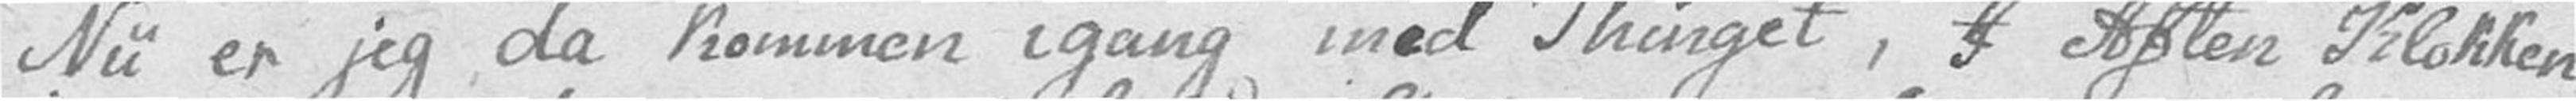Nu er jeg da kommen igang med Thinget, I Aften Klokken
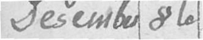Desember 8te
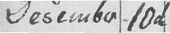Desember 10de
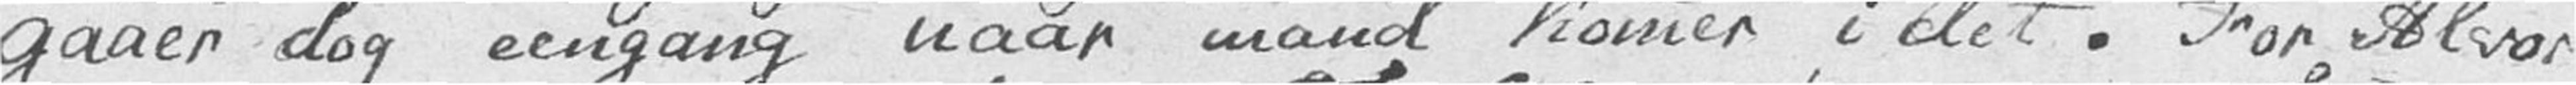gaaer dog eengang naar mand komer i det. For Alvor
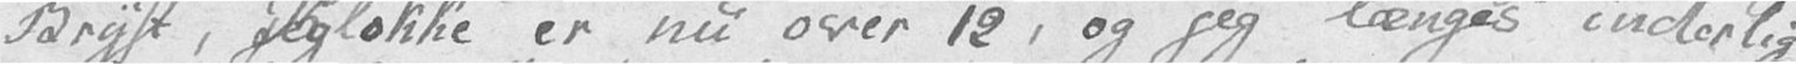Bryst, Klokke er nu over 12, og jeg længes inderlig
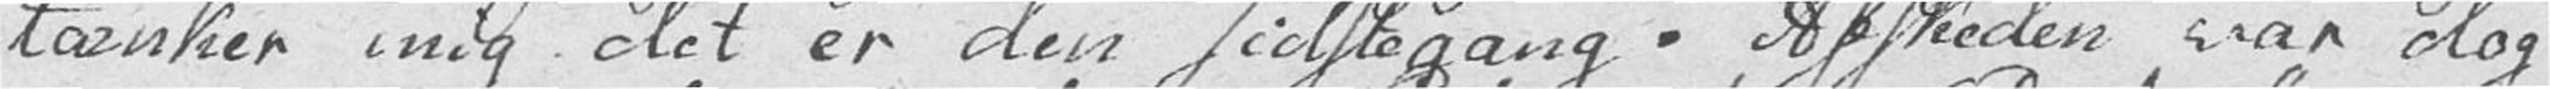tænker mig det er den sidstegang. Afskeden var dog
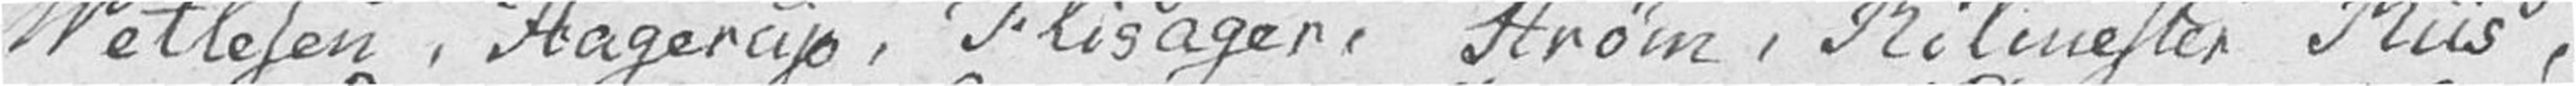Vetlesen, Hagerup, Flisager, Strøm, Ritmester Riis,
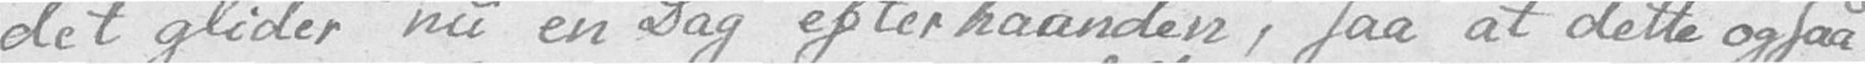det glider nu en Dag efterhaanden, saa at dette ogsaa
